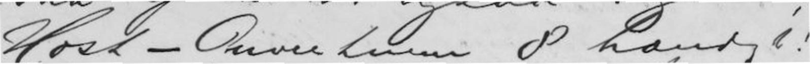Høst-Ouverturen 8 hændigt!
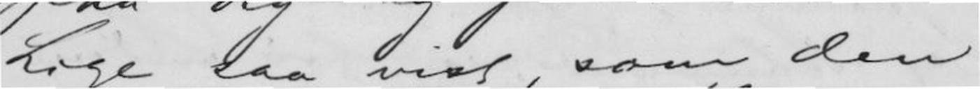Lige saa vist, som den
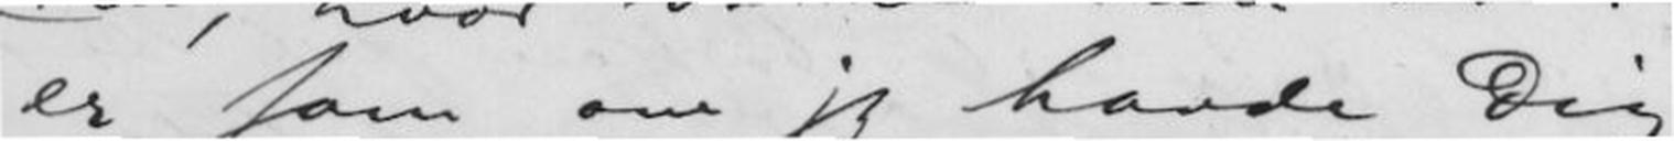er som om jeg havde Dig
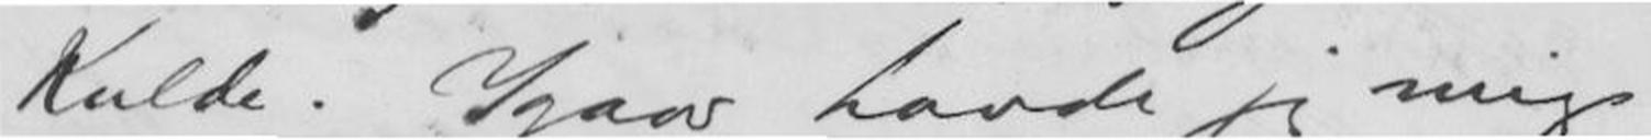Kulde. Igaar havde jeg mig
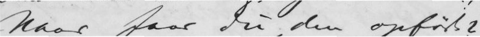Naar faar du den opført?
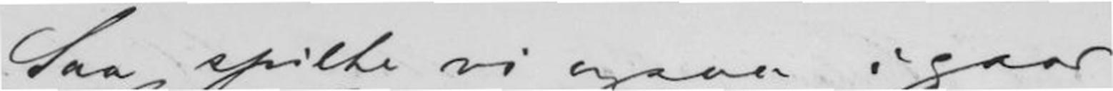Saa spilte vi ogsaa igaar,
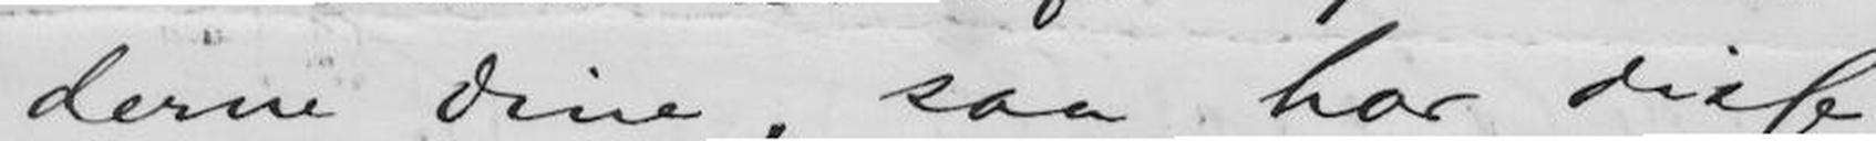derne dine, saa har disse
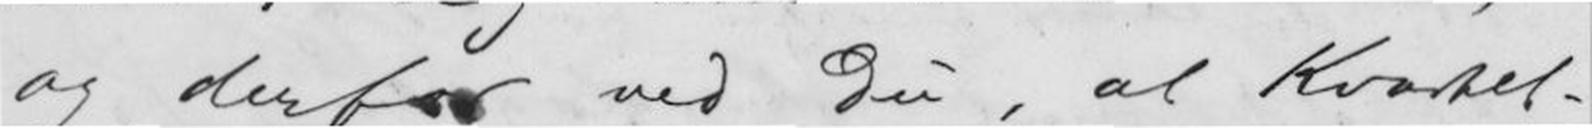og derfor ved Du, at Kvartet-
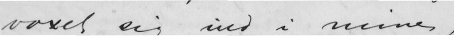voxet sig ind i mine,
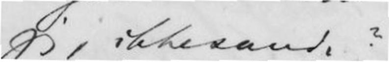jeg, ikkesandt?
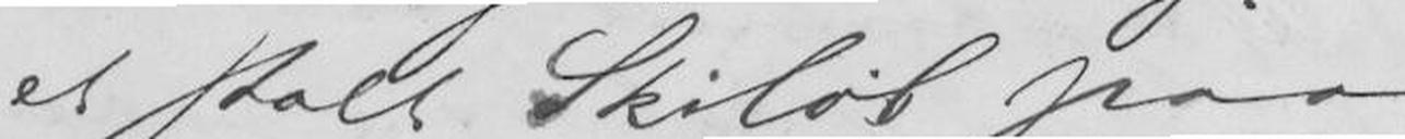et stolt Skiløb paa
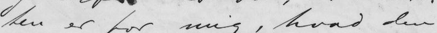ten er for mig, hvad den
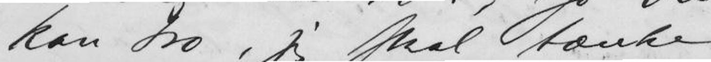kan tro, jeg skal tænke
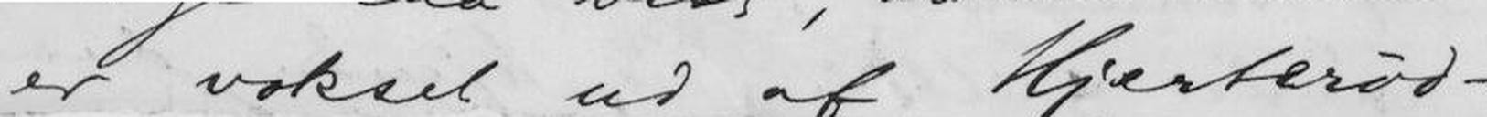er vokset ud af Hjerterød-
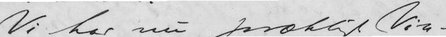Vi har nu præktigt Vin-
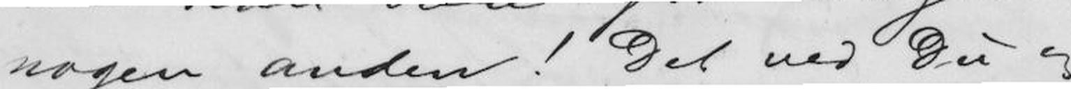nogen anden! Det ved Du og
